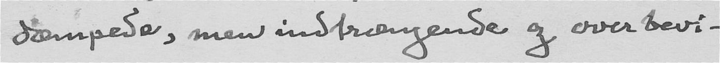dæmpede, men indtrængende og overbevi-
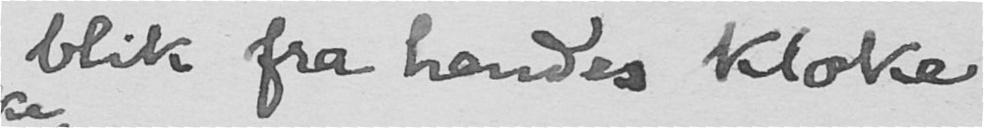blik fra hendes kloke
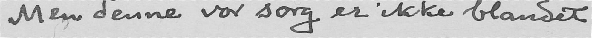Men denne vor sorg er ikke blandet
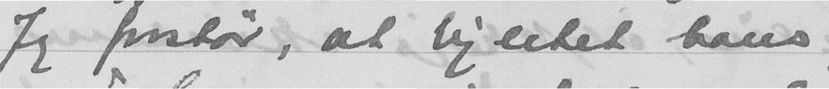Jeg forstår, at hjertet hans
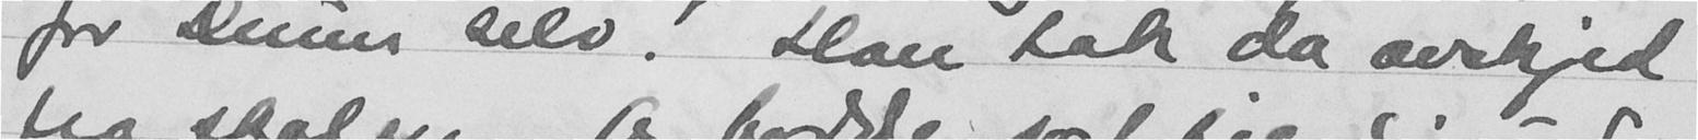for Duun selv! Han tok da avskjed
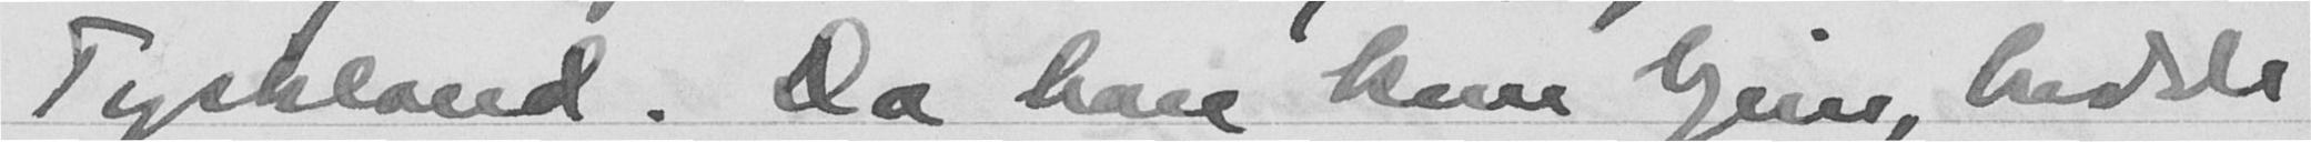Tyskland. Da han kom hjem hadde
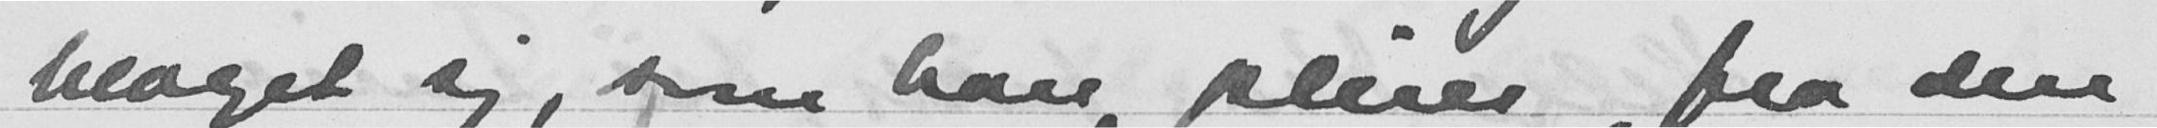klaget sig, som han pleier, fra den
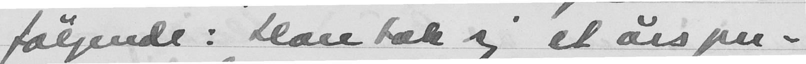følgende: Han tok sig et års per-
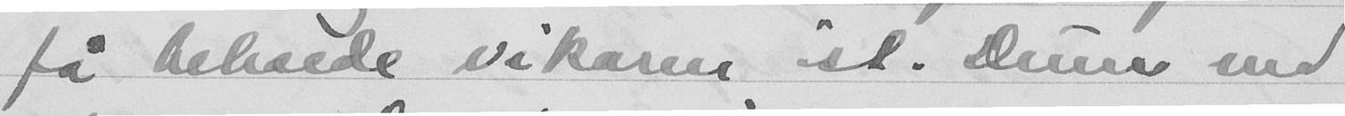få beholde vikaren ut. Duun ved
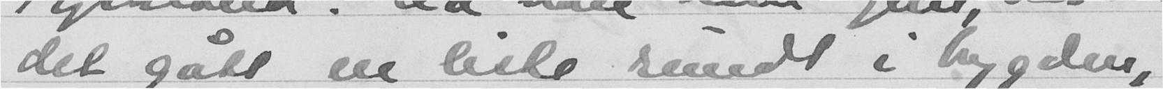det gått en liste rundt i bygden,
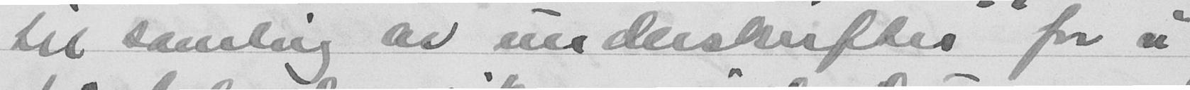til samling av underskrifter for å
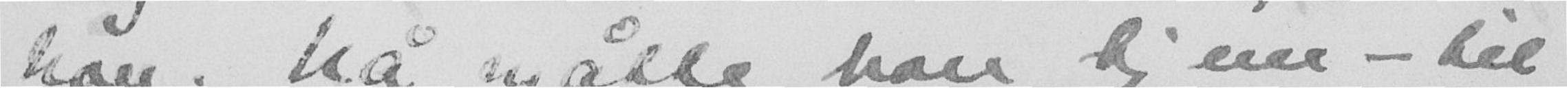han. Nå måtte han hjem - til
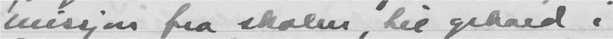misjon fra skolen, til ophold i
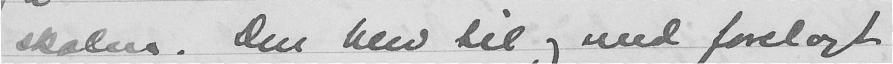skolen. Den blev til og med forelagt
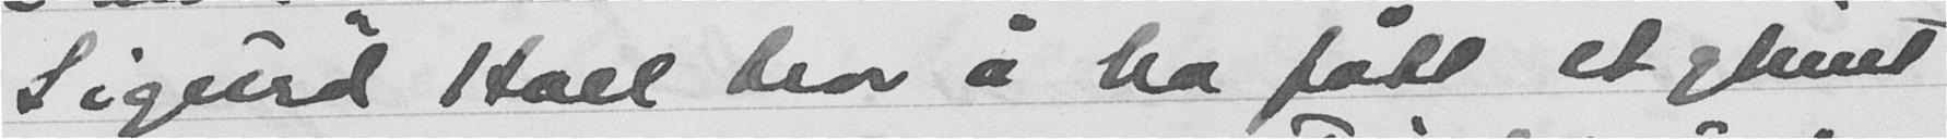Sigurd Hoel tror å ha fått et glimt
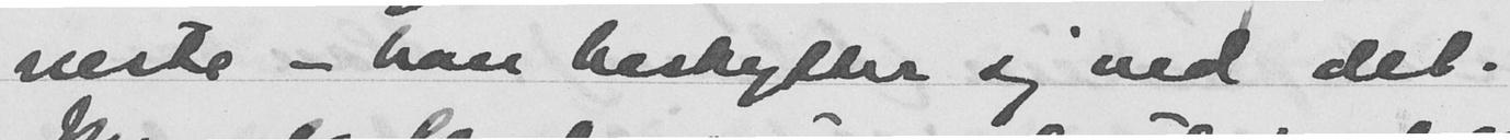neste og han beskytter sig ved det.
